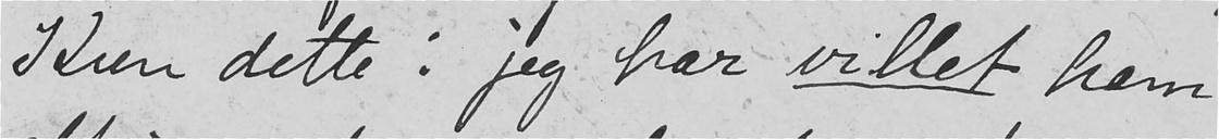Kun dette: jeg har villet ham
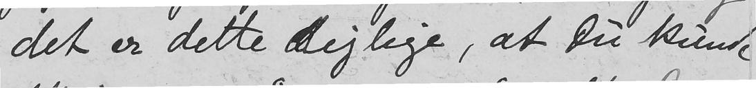det er dette dejlige, at Du kunde
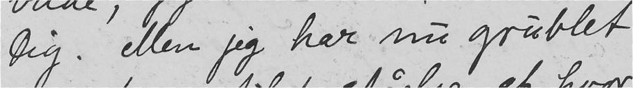Dig. Men jeg har nu grublet
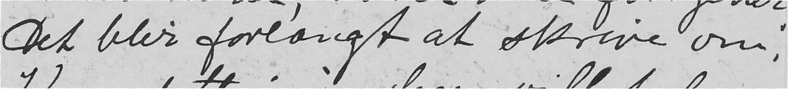Det blir forlangt at skrive om.
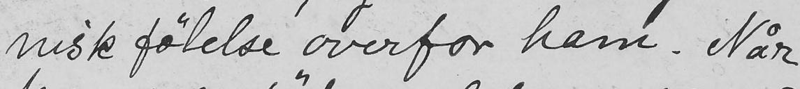nisk følelse overfor ham. Når
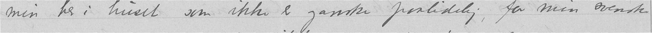min her i huset som ikke er ganske paalidelig, for min svenske
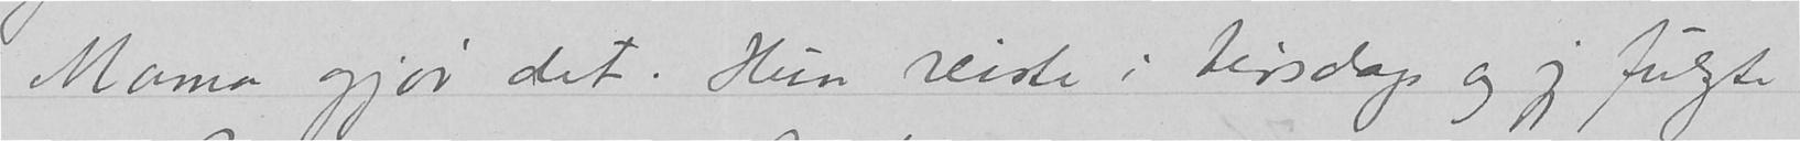Mama gjør det. Hun reiste i tirsdag og jeg fulgte
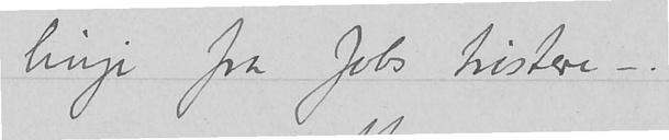linge fra Jobs tristere –.
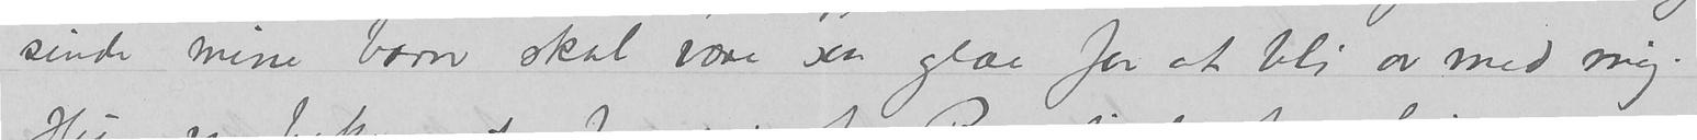sinde mine barn skal være saa glae for at bli av med mig.
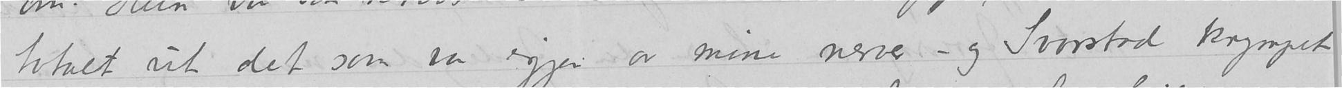totalt ut det som var igjen av mine nerver – og Svarstad krympet
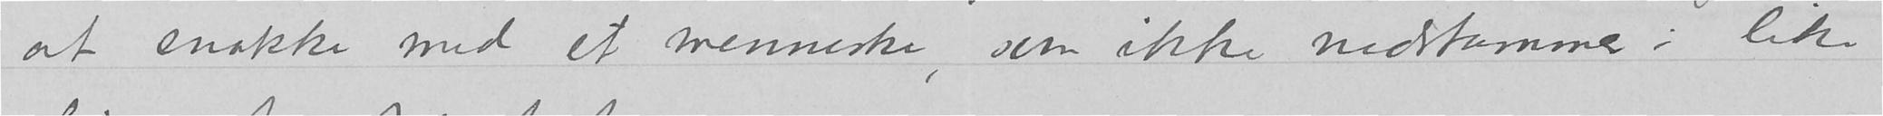at snakke med et menneske, som ikke nedstammer i like
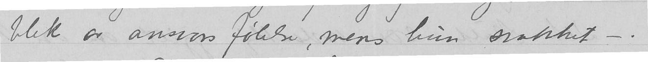blek av ansvarsfølelse mens hun snakket –
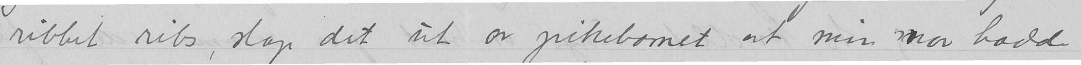ribbet ribs, slap det ut av pikebarnet at min mor hadde
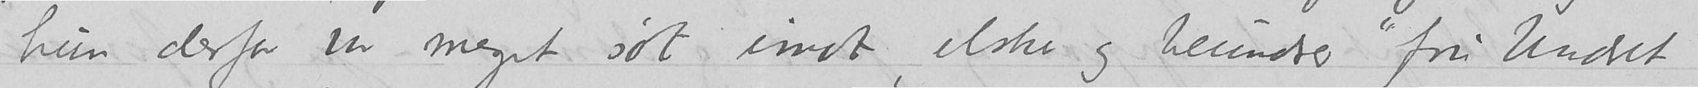hun derfor var meget søt imot elske og beundrer «fru Undset»
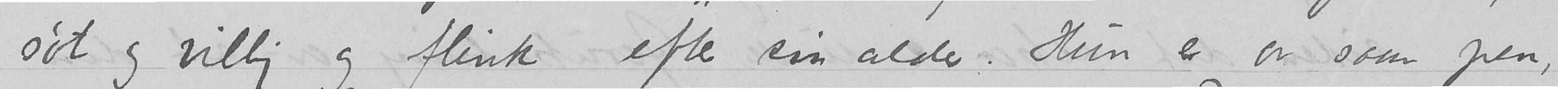søt og villig og flink efter sin alder. Hun er av saan pen,
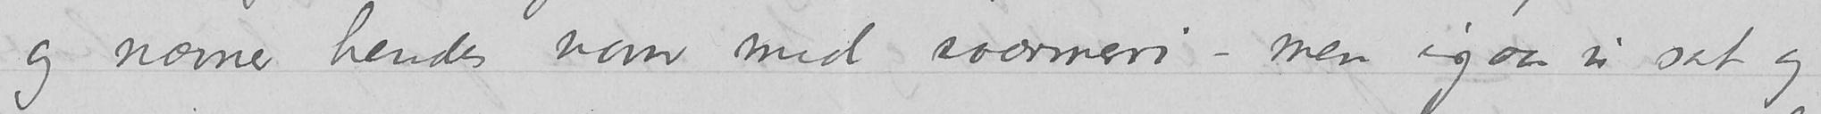og nævner hendes navn  med sværmeri – men igaar vi sat og
